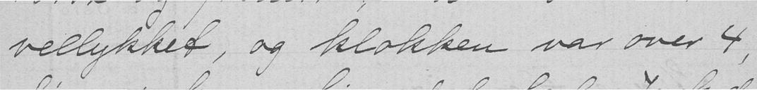vellykket, og klokken var over 4,
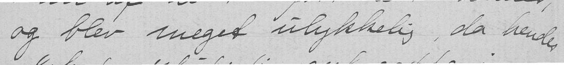og blev meget ulykkelig, da hendes
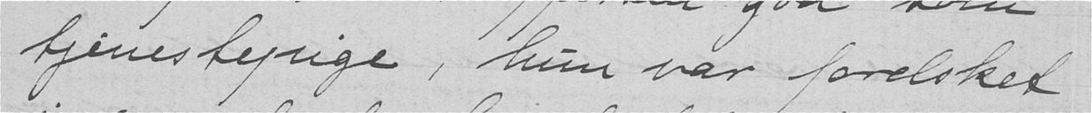tjenestepige, hun var forelsket
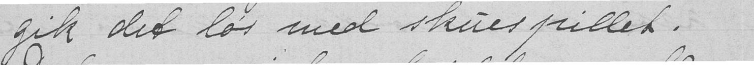gik det løs med skuespillet.
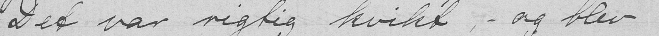Det var rigtig kvikt ,- og blev
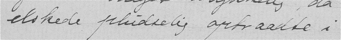elskede pludselig optraadte i
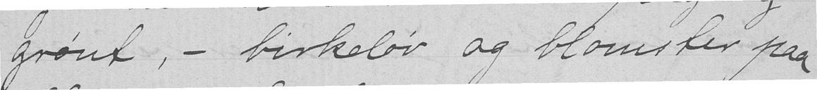grønt, - birkeløv og blomster paa
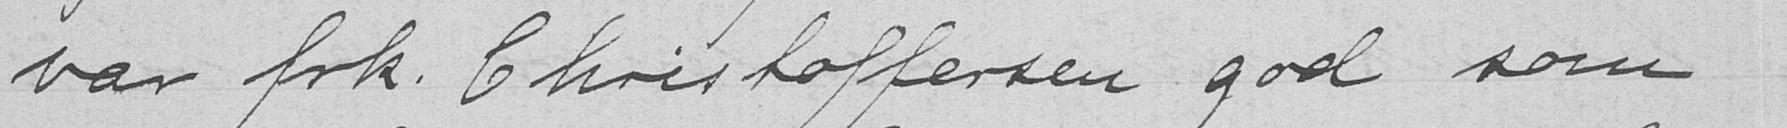var frk. Christoffersen god som
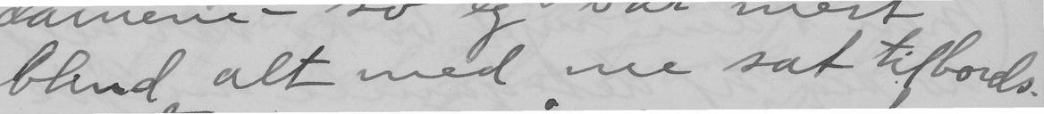blind alt med me sat tilbords.
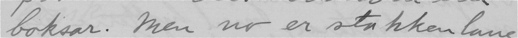boksar. Men no er stakken lang
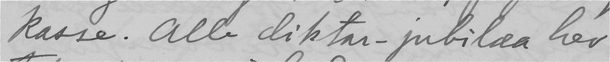kasse. Alle diktar-jubilæa hev
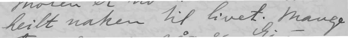heilt naken til livet. Mange
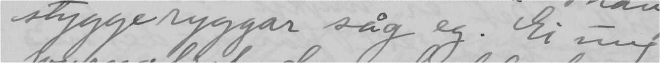stygge ryggar såg eg. Ei ung
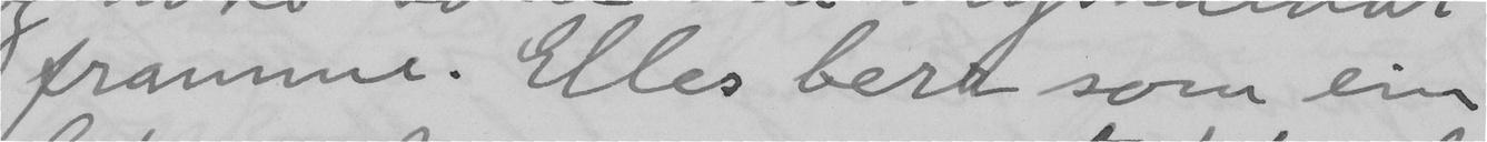framme. Elles bera som ein
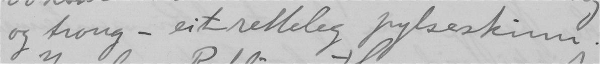og trong – eit retteleg pylseskinn.
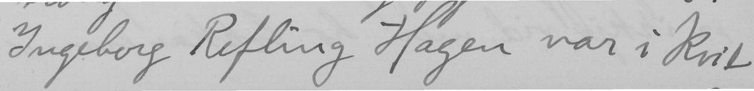Ingeborg Refling Hagen var i kvit
